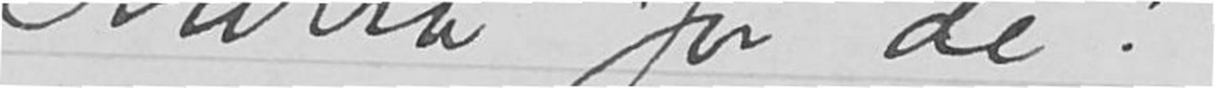Hurra for de!
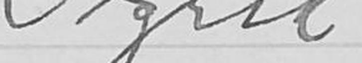Sigrid.
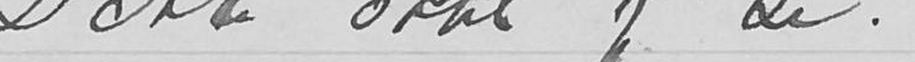Dette skal jeg se!
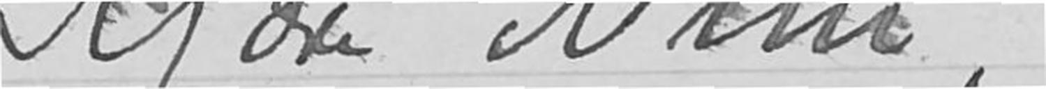Kjære Nini,
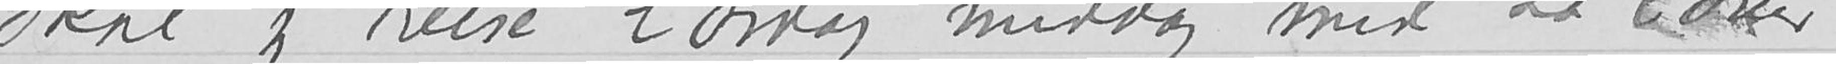skal jeg reise lørdag middag med La Cou<e>r
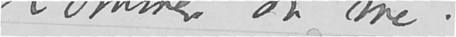Kommer du me?
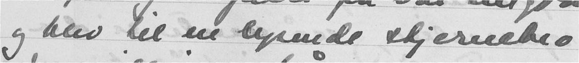og blev til en lysende stjernebro
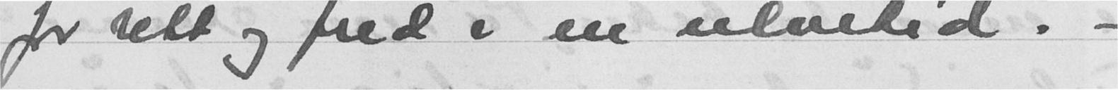for rett og fred i en ulvetid. -
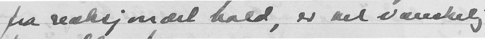fra reaksjonært hold, er vel vanskelig
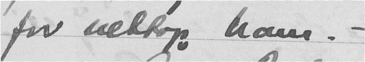for nettop ham. -
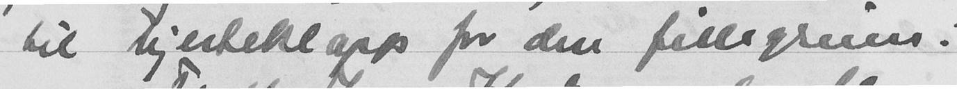til hjerteklapp for den fillegreien.
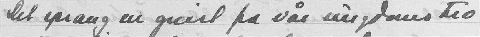Det sprang en gnist fra var ungdoms tro
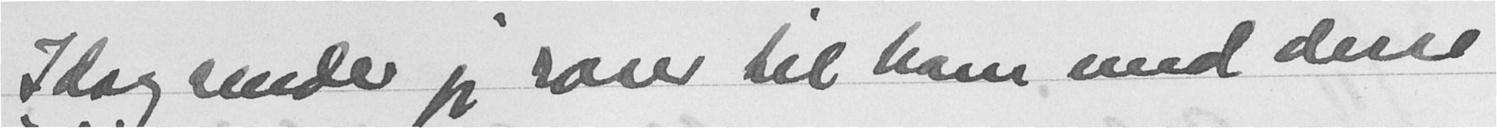Idag sender jeg roser til ham med disse
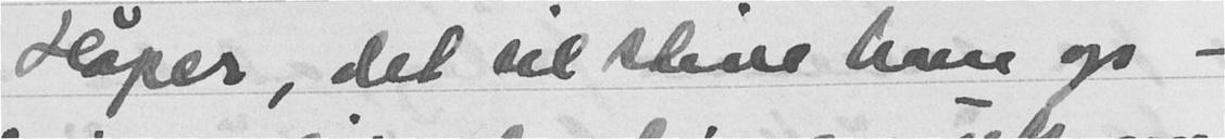Håper, det vil stive ham op -
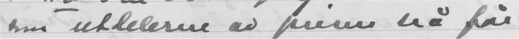som utdelerne av prisen nå får
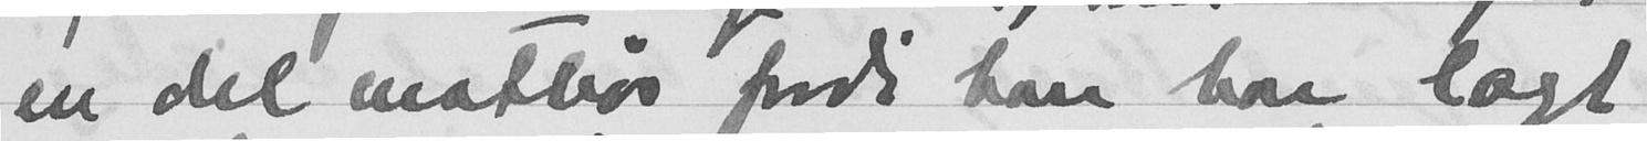en del motbør fordi han har lagt
